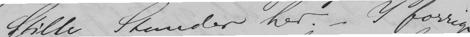stille Stunder her. - I forrige
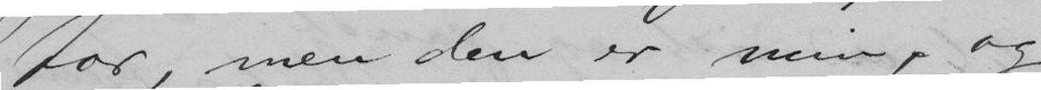tor, men den er min, og
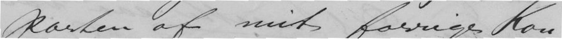parten af mit forrige Kon-
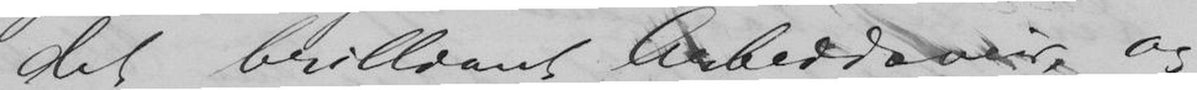det brilliant Arbeidsveir, og
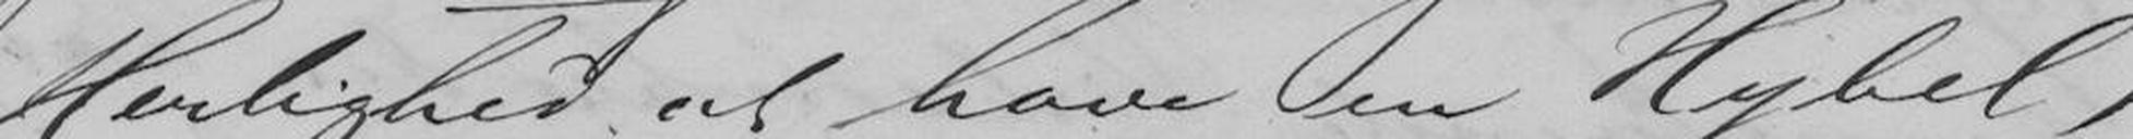Herlighed at have en Hybel,
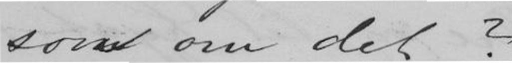son om det?
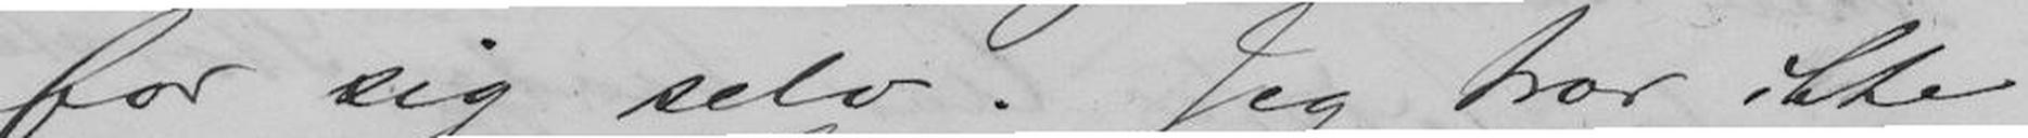for sig selv. Jeg tror ikke
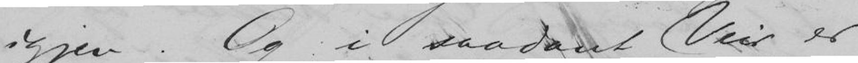igjen. Og i saadant Veir er
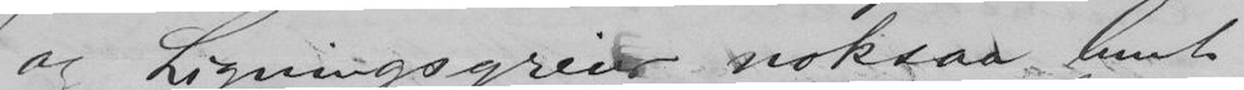og Ligningsgreier noksaa lunt
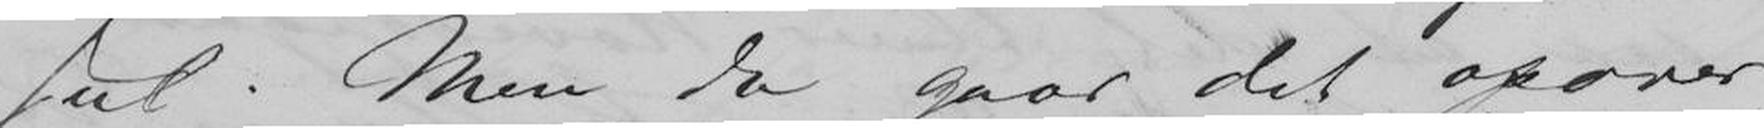Jul. Men da gaar det opover
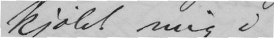kjølet mig i
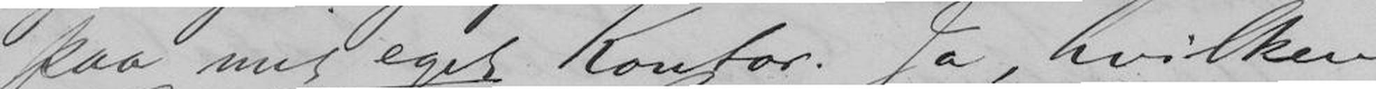paa mit eget Kontor. Ja, hvilken
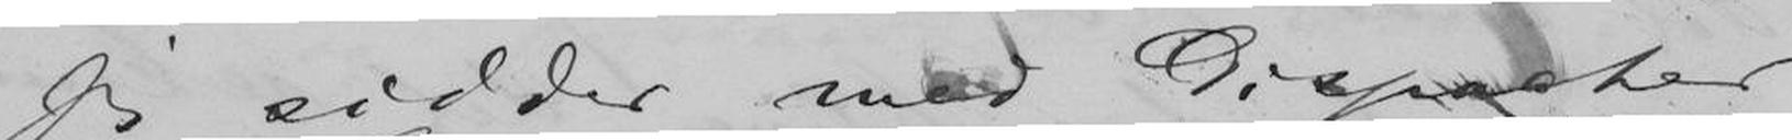jeg sidder med Dispacher
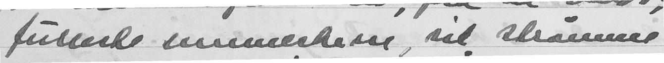fulleste menneskene, vil strømme
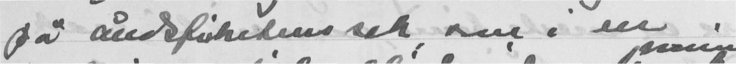på åndsfrihetens sak, som i en
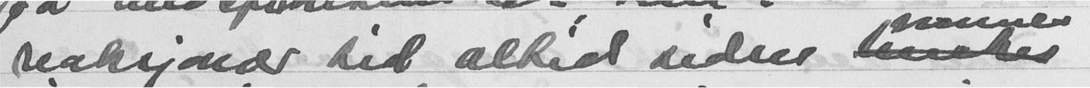reaksjonær tid altid siden  somnen
